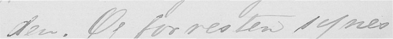den. Og forresten synes
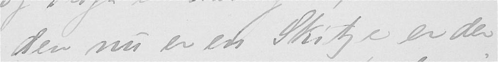den nu er en Skitze er der
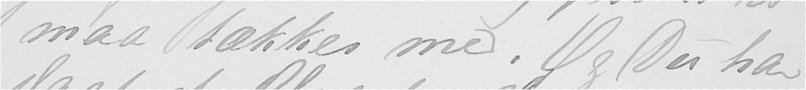maa tækkes med. Og Du har
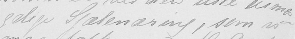gelige Sjælenæring, som vi
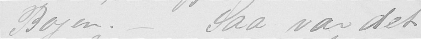Bogen. Saa var det
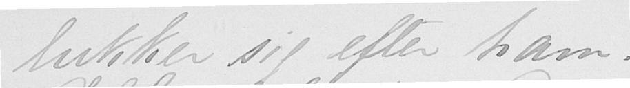lukker sig efter ham.
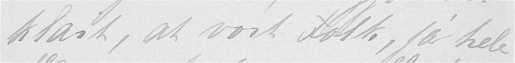klart, at vort Folk, ja hele
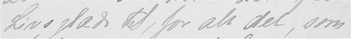Livsglæde til, for alt det, som
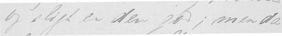og sligt er den god; men da
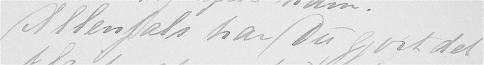Allenfals har Du giort det
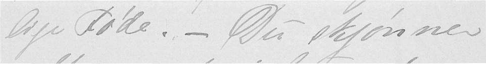lige Føde. - Du skjønner
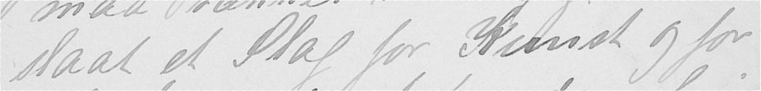slaat et Slag for Kunst og for
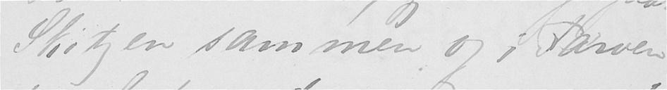Skitzen sammen og i Farver
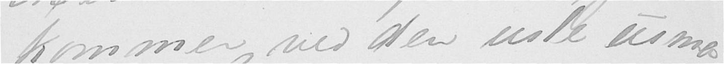kommer ved den usle usma-
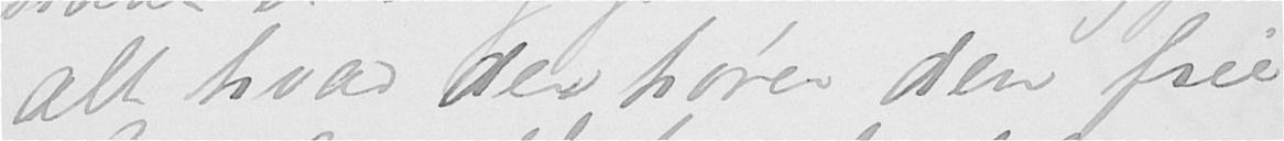alt hvad der hører den frie
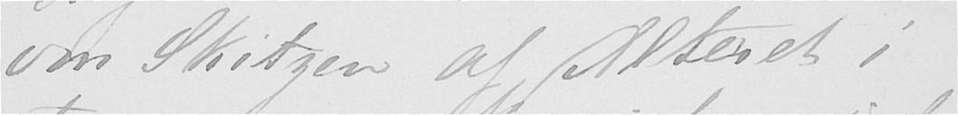om Skityen af Alteret i
